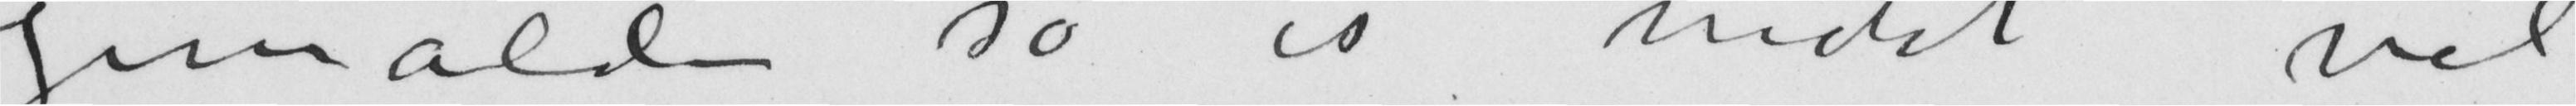Gemalden so es nicht vil
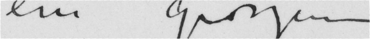ein groszen
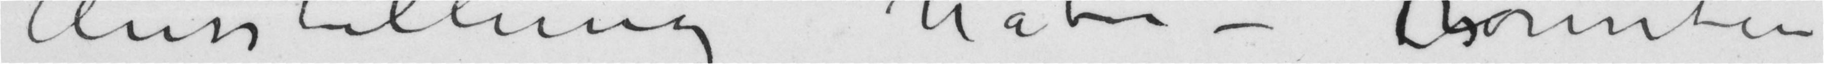Ausstellung habe – EsKonnten
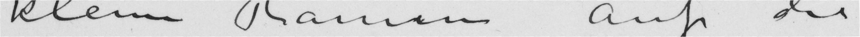kleine Ramen auf den
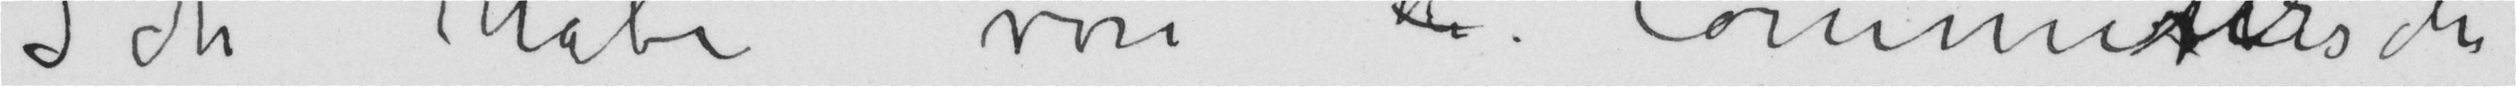Ich habe von Hr. Commerttersche
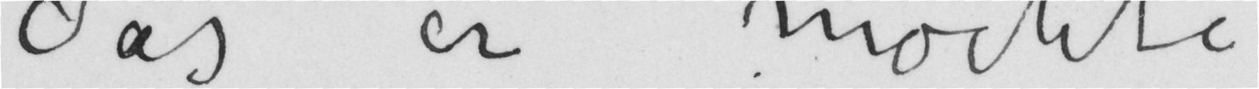das er möchte
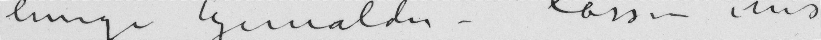einige Gemalden – lassen uns
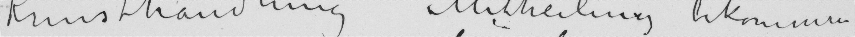Kunsthandlung Mitheilung bekommen
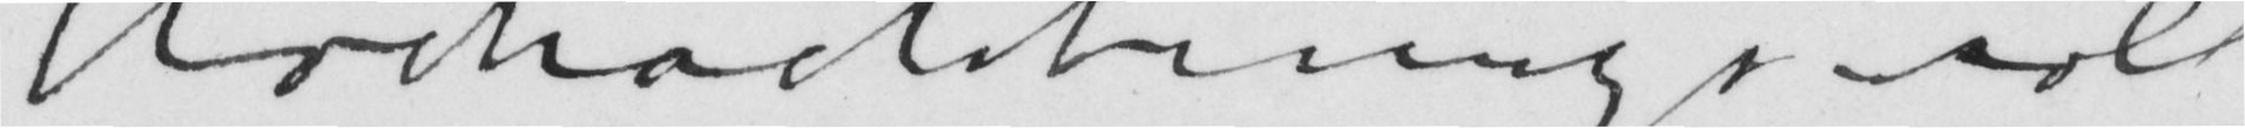Hochachtungsvoll
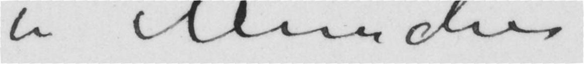in München
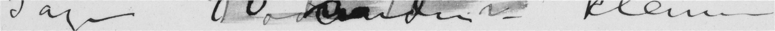sagen 7 oder 10 s10 senden – kleinen
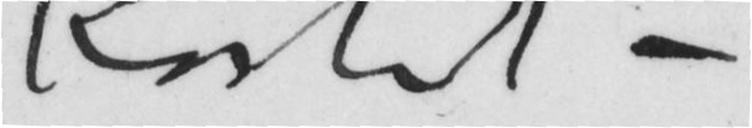kostet –
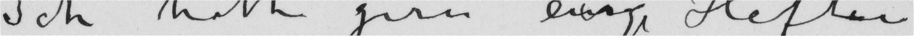Ich hatte gern einige Heften
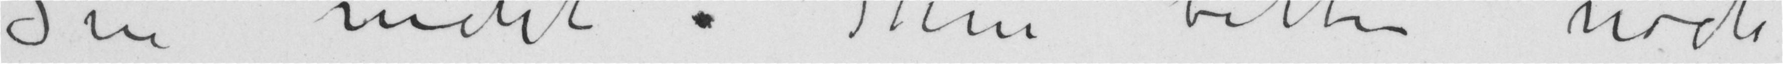Sie nicht Ihm bitten noch
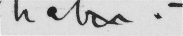habene
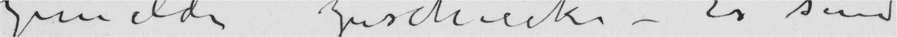Gemalde zuschiecke – Es sind
